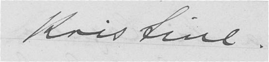Kristine.
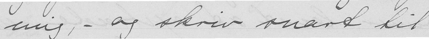mig, - og skriv snart til
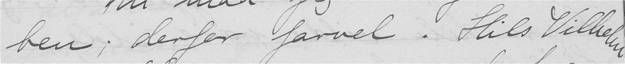ben; derfor farvel. Hils Vilhelm
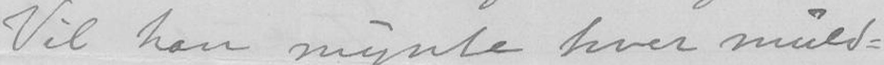Vil han mynte hver muld-
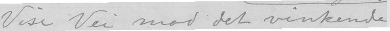Vise Vei mod det vinkende
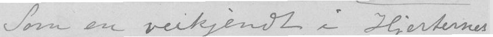Som en veikjendt i Hjertenes
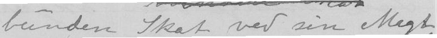bunden Skat ved sin Magt,
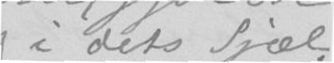i dets Sjæl,
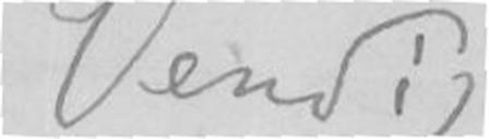(Vend!)
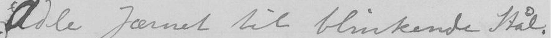Ædle Jærnet til blinkende Stål
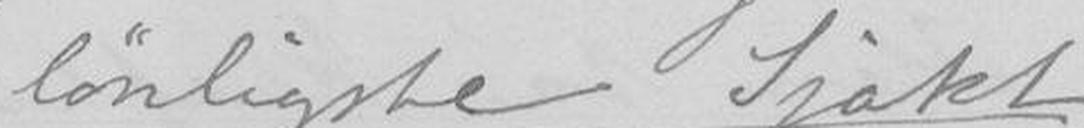lønligste Sjakt
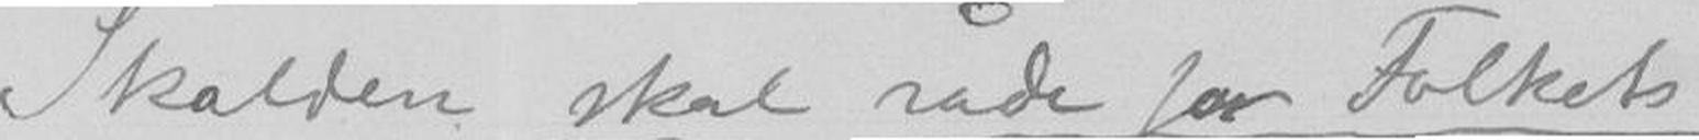Skalden skal råde for Folkets
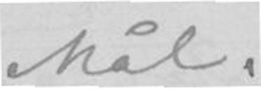Mål.
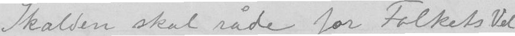Skalden skal råde for Folkets Vel!
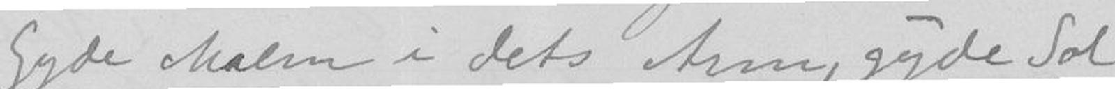Gyde Malm i dets Arm, gyde Sol
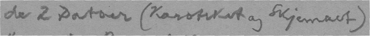de 2 Datoer (Karoteket og Skjemaet)
